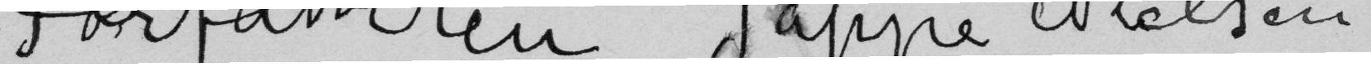Forfatteren Jappe Nielsen
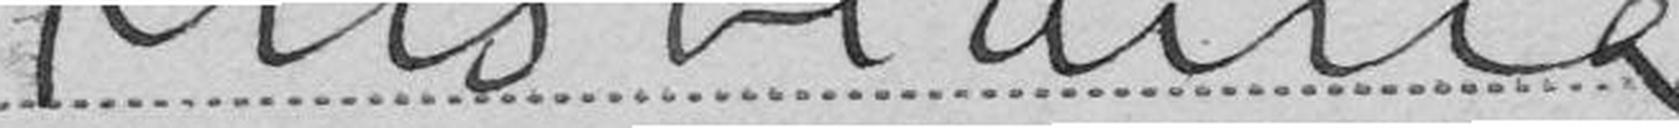Kristiania
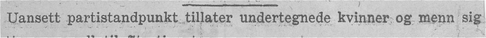Uansett partistandpunkt tillater undertegnede kvinner og menn sig
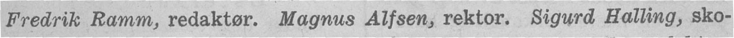Fredrik Ramm, redaktør. Magnus Alfsen, rektor. Sigurd Halling, sko-
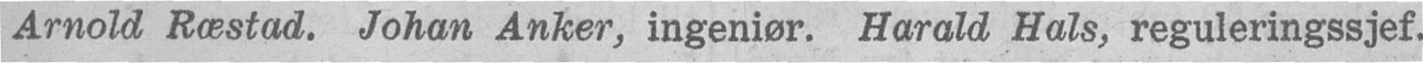Arnold Ræstad. Johan Anker, ingeniør. Harald Hals, reguleringssjef.
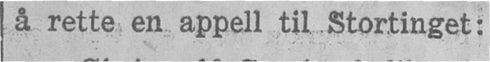å rette en appell til Stortinget:
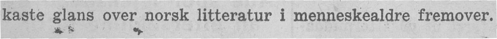kaste glans over norsk litteratur i menneskealdre fremover.
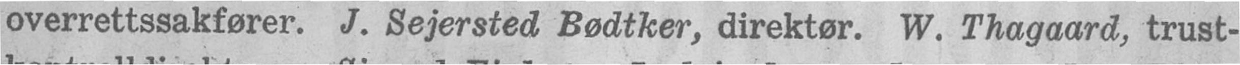overrettssakfører. J. Sejersted Bødtker, direktør. W. Thagaard, trust-
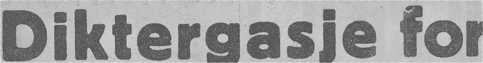Diktergasie for
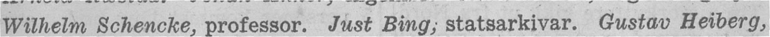Wilhelm Schencke, professor. Just Bing, statsarkivar. Gustav Heiberg,
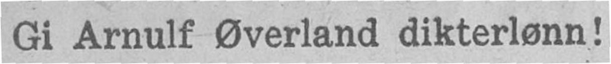Gi Arnulf Øverland dikterlønn!
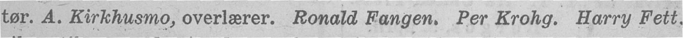tør. A. Kirkhusmo, overlærer. Ronald Fangen. Per Krohg. Harry Fett.
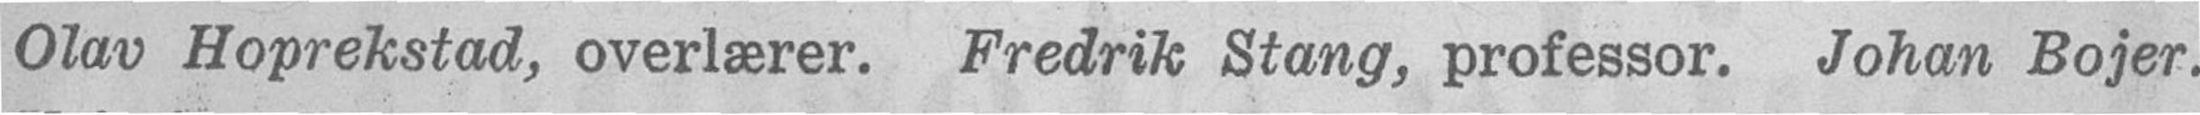Olav Hoprekstad, overlærer. Fredrik Stang, professor. Johan Bojer.
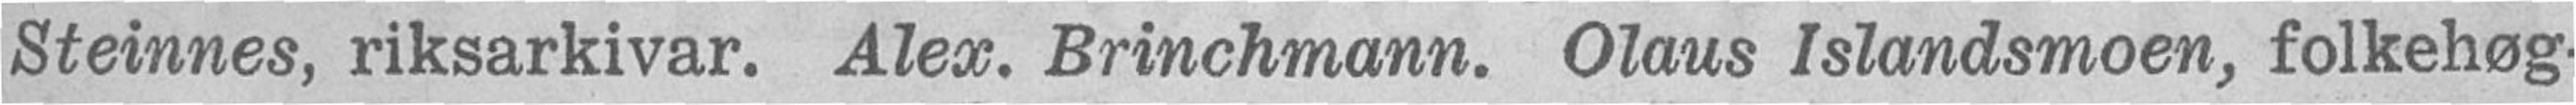Steinnes, riksarkivar. Alex. Brinchmann. Olaus Islandsmoen, folkehøg-
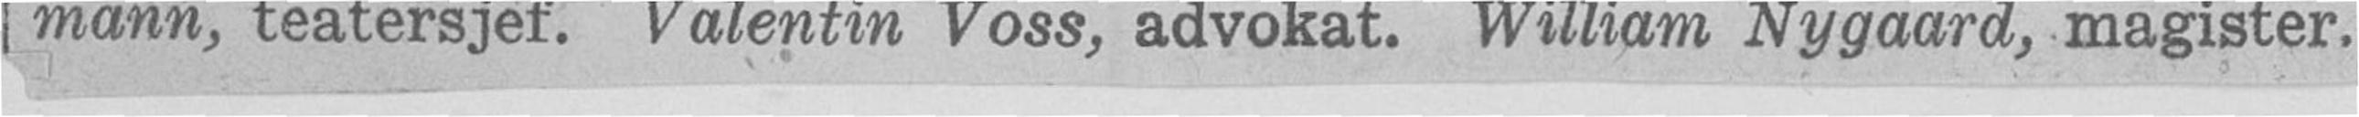mann, teatersjef. Valentin Voss, advokat. William Nygaard, magister.
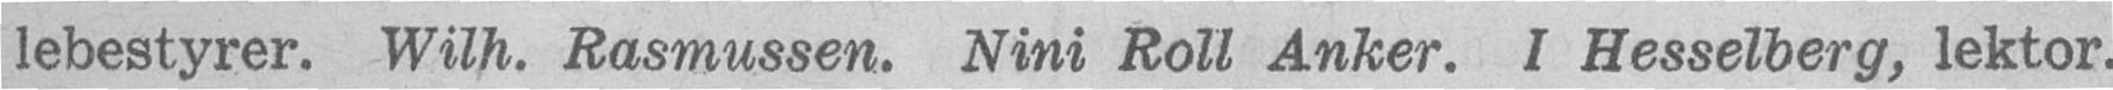lebestyrer. Wilh. Rasmussen. Nini Roll Anker. I Hesselberg, lektor.
